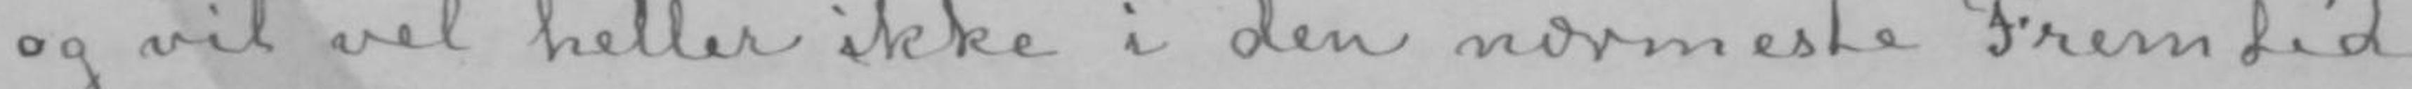og vil vel heller ikke i den nærmeste Fremtid
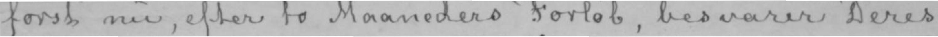først nu, efter to Maaneders Forløb, besvarer Deres
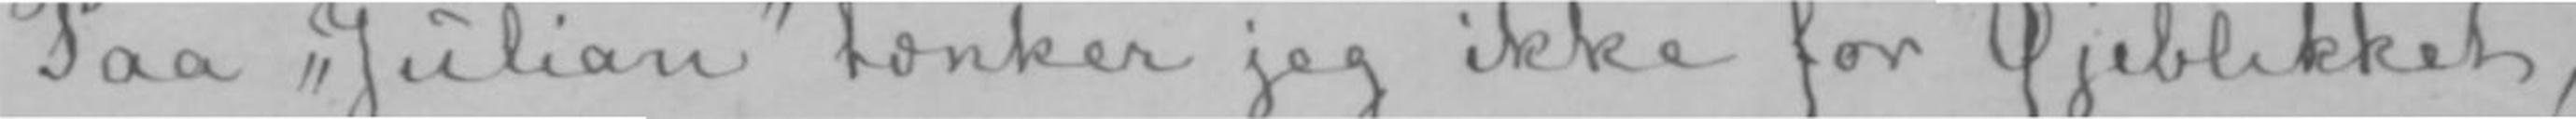Paa «Julian» tænker jeg ikke for Øjeblikket,
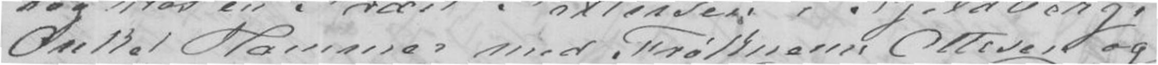Onkel Hammer med Frøknerne Ottesen og
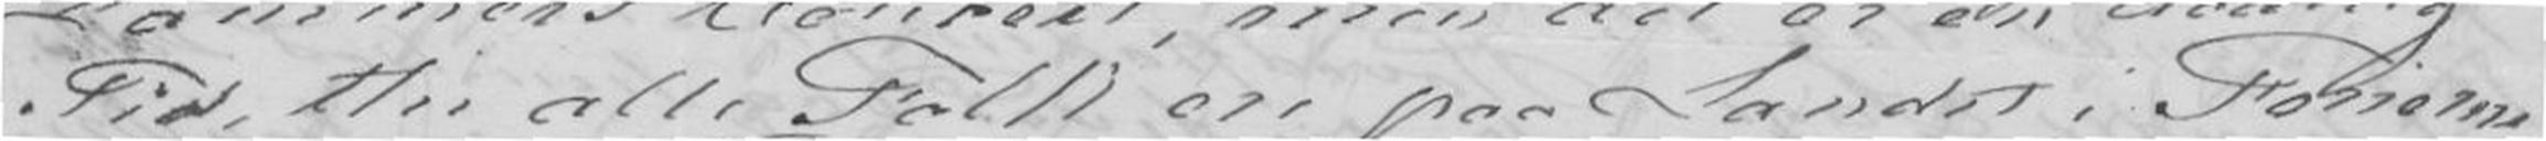Tid, thi alle Folk ere paa Landet i Feriene
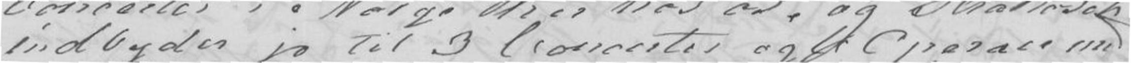indbyder jo til 3 Concerter og 6 Operaer med
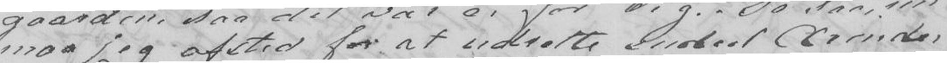maa jeg afsted for at udrette endeel Ærinder
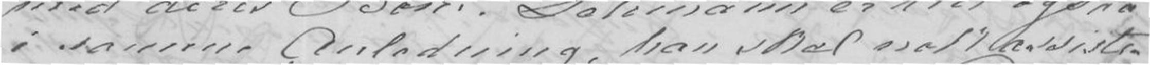i samme Anledning, han skal nok assiste-
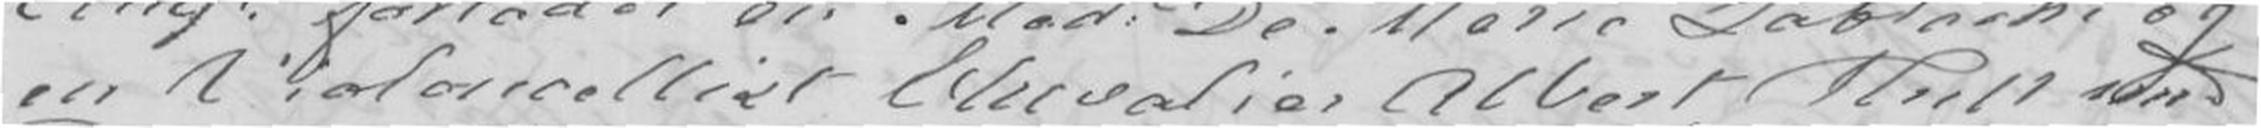en Violoncellist Chevalier Albert Hull med
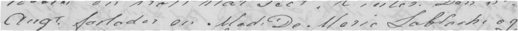Augt. forlader en Mad: De Marie Lablache og
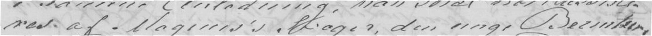res af Magnus's Svoger, den unge Berentzen
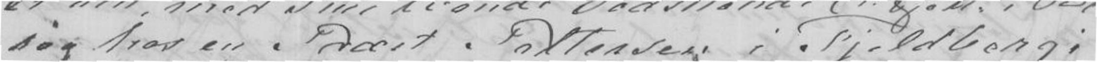søg hos en Præst Pettersen i Fjeldberg;
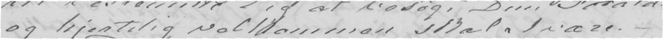og hjertelig velkommen skal I være. -
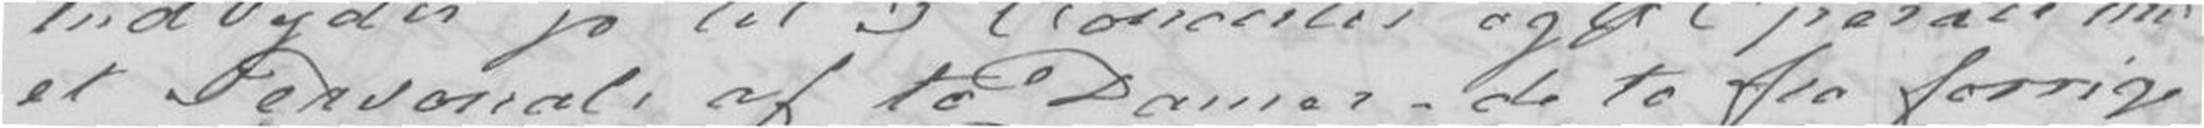et Personale af to Damer - de to fra forrige
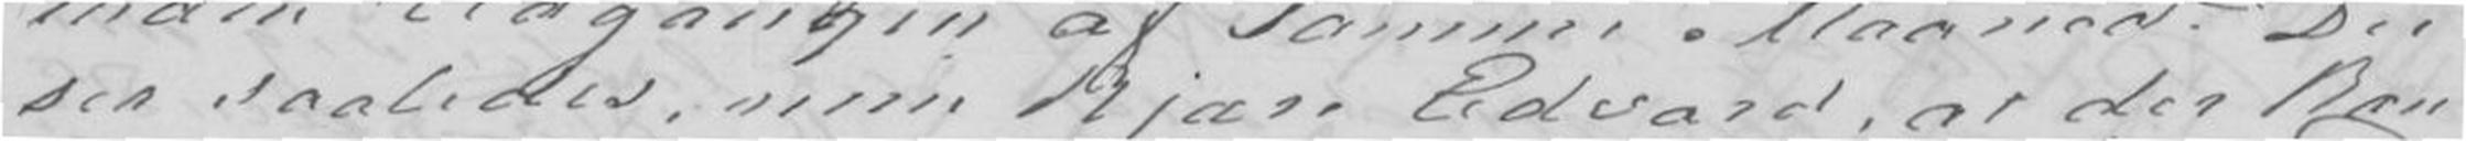ser saaledes, min kjære Edvard, at der kan
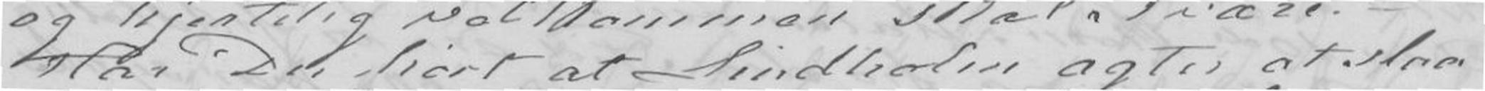Har Du hørt at Lindholm agter at slaa
